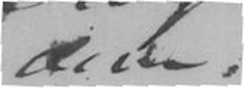dem.
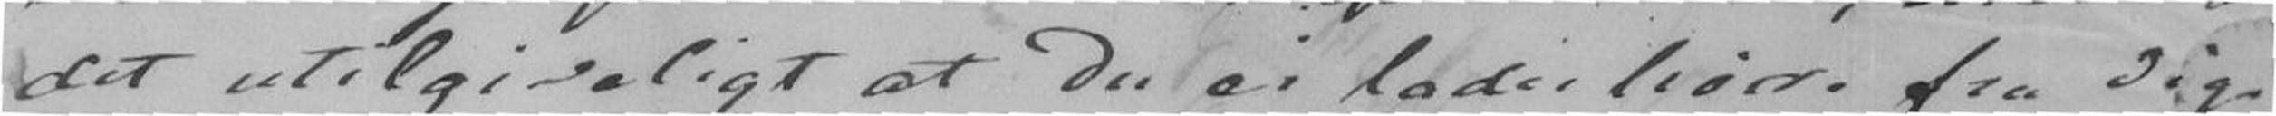det utilgiveligt at Du ei lader høre fra Dig.
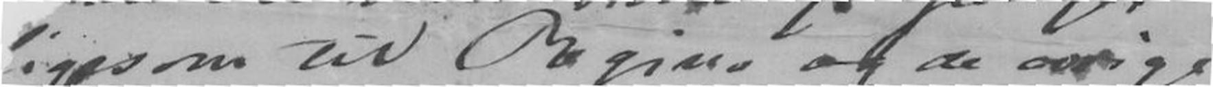ligesom til Regina og de øvrige
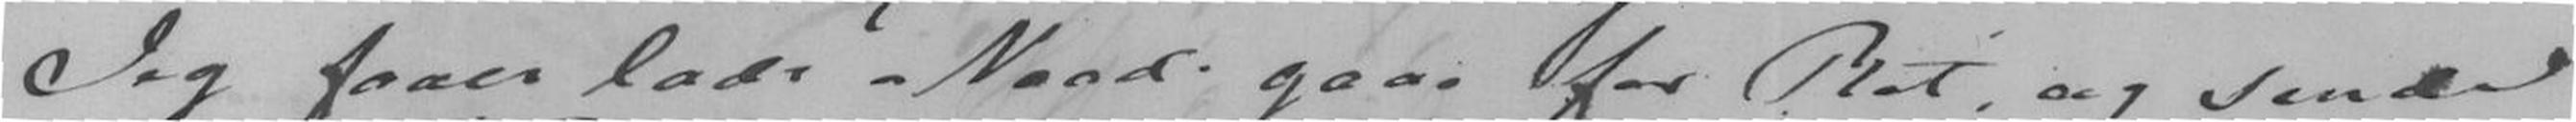Jeg faaer lade Naade gaae for Ret, og sender
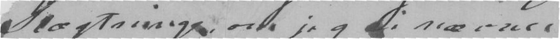Slægtninger om jeg ei nævner
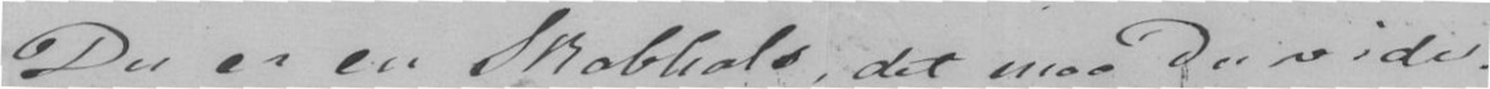Du er en Skabhals, det maa Du vide.
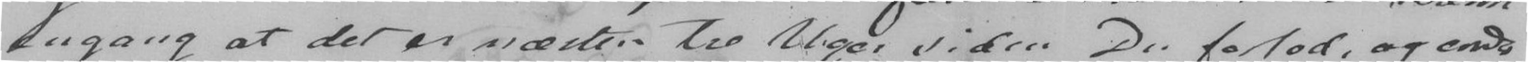engang at det er tre Uger siden Du forlod, og end-
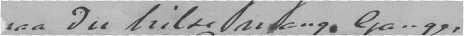maa Du hilse mange Gange
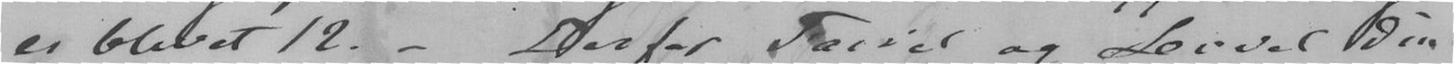er blevet 12. - Derfor Farvel og Levvel Din
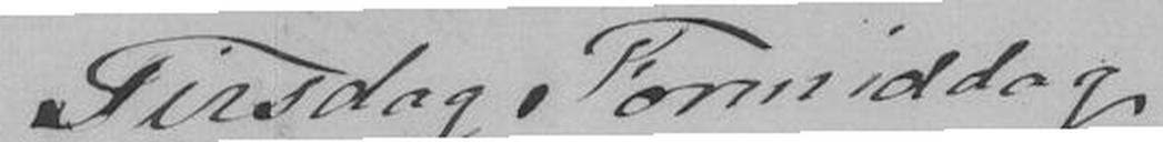Tirsdag Formiddag
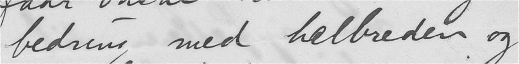bedring med helbreden og
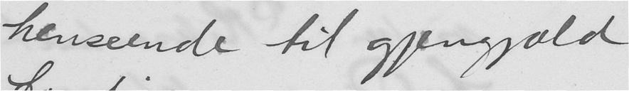henseende til gjengjæld
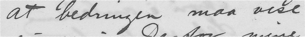at bedringen maa vise
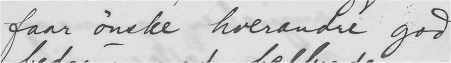faar ønske hverandre god
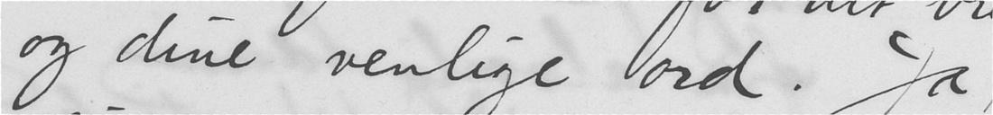og dine venlige ord. Ja,
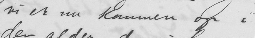vi er nu kommen op i
